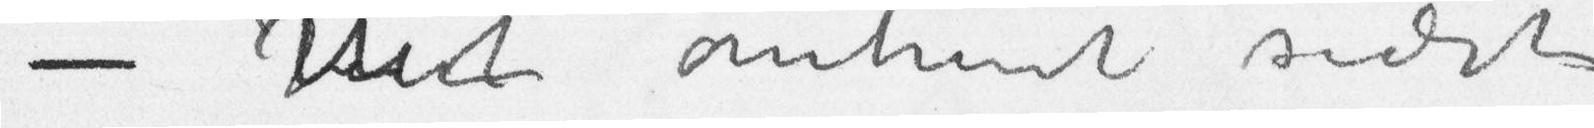– Mit omtrent sidste
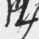12)
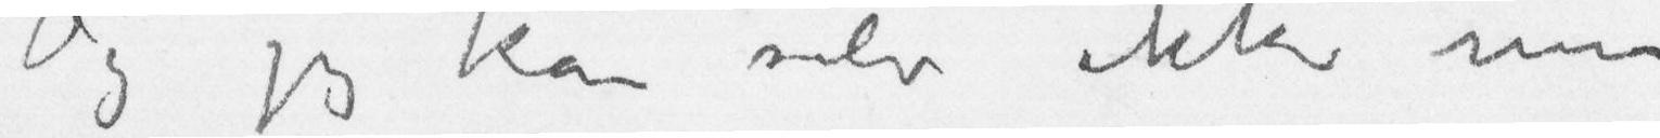Og jeg kan selv ikke mer
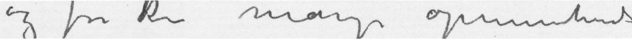og for de mange opmuntrende
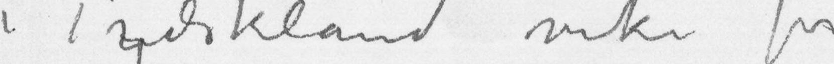i Tydskland virke for
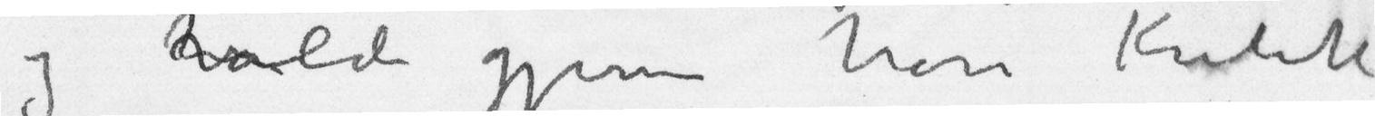og vilde gjerne have Kritik
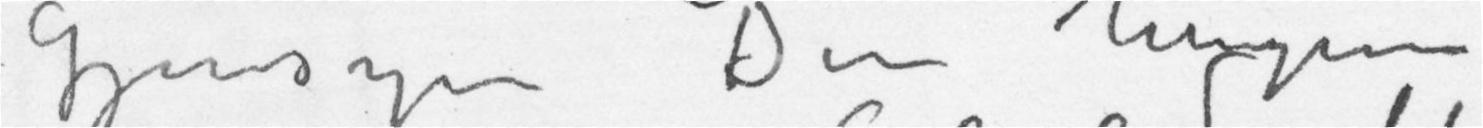GjensynDin hengivne
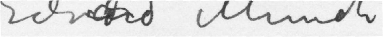Edvard Munch
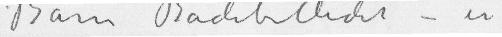Barn Badebilledet – er
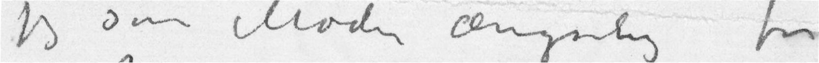jeg som Moder ængstelig for
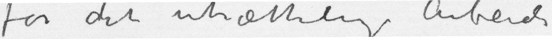for dit utrættelige Arbeide
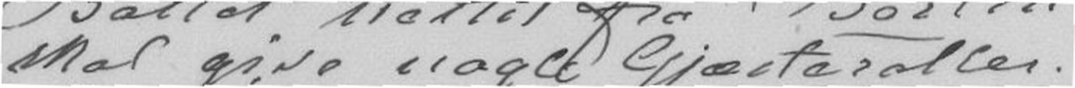skal give nogle Gjæsteroller.
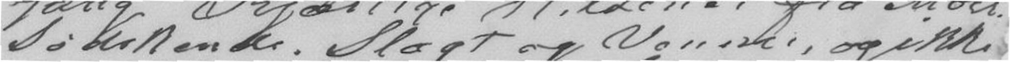Sødskende, Slægt og Venner, og ikke
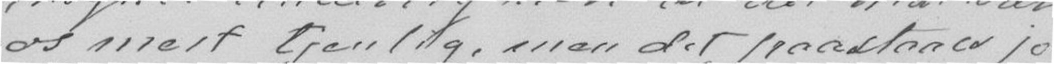os mest tjenlig, men det paastaaes jo
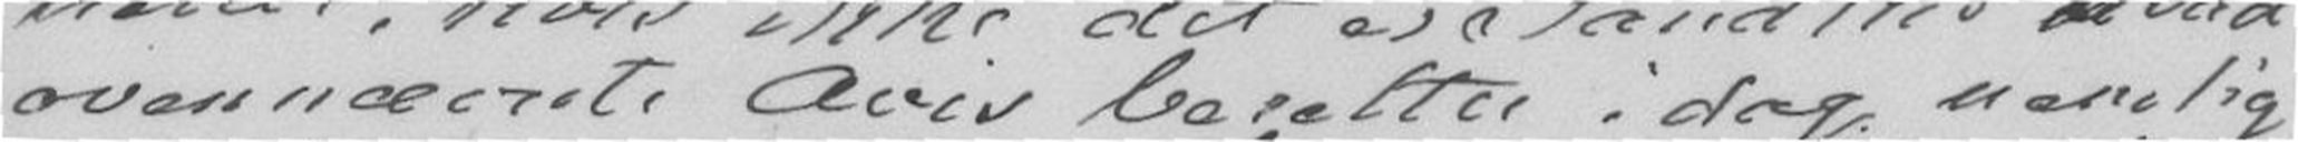overnævnte Avis berætter idag: nemlig
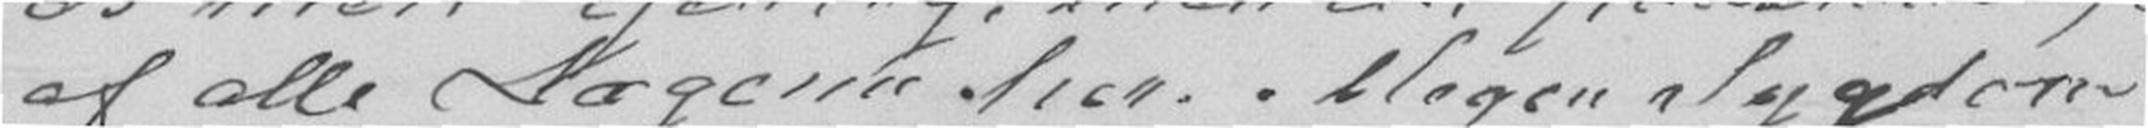af alle Lægene her. Megen Sygdom
